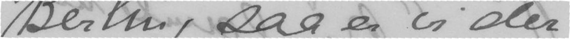Berlin, saa er vi der.
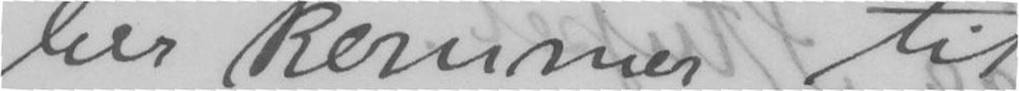ber kommer til
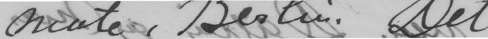møte i Berlin. Det
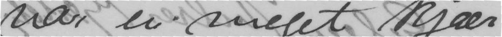var en meget kjær
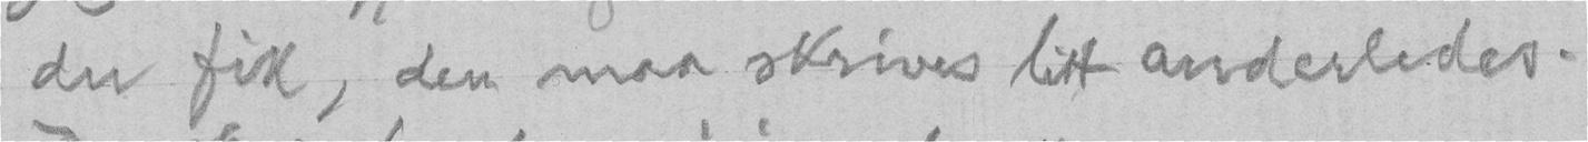du fik, den maa skrives litt anderledes.
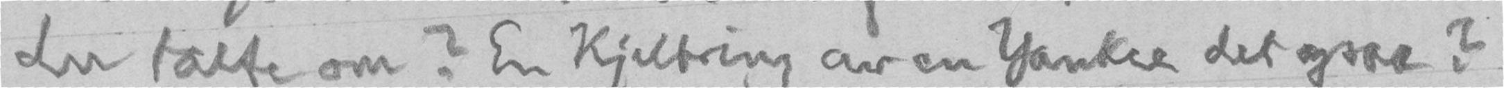du talte om? En Kjeltring av en Yankee det ogsaa?
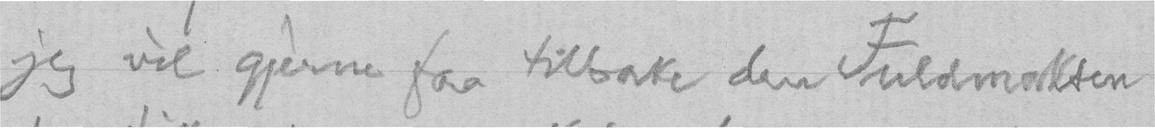jeg vil gjerne faa tilbake den Fuldmakten
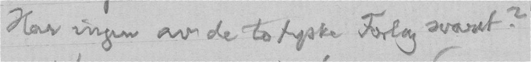Har ingen av de to tyske Forlag svaret?
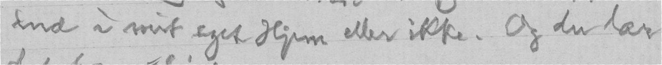ind i mit eget Hjem eller ikke. Og du lar
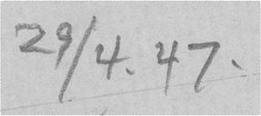29/4. 47.
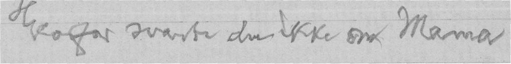Hvorfor svarte du ikke om Mama
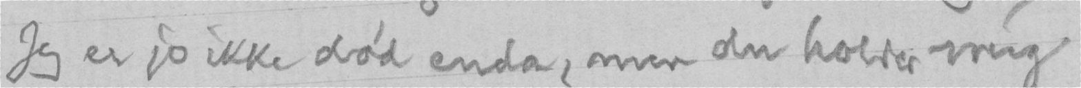Jeg er jo ikke død enda, men du holder mig
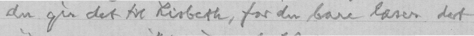du gir det til Lisbeth, for du bare læser det
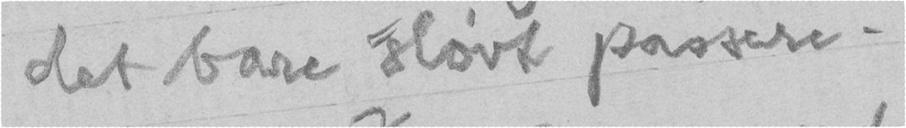det bare sløvt passere.
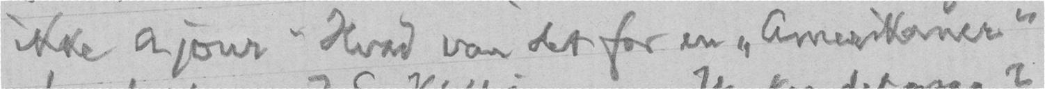ikke ajour? Hvad var det for en "Amerikaner"
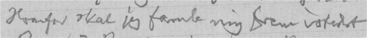Hvorfor skal jeg famle mig frem istedet
